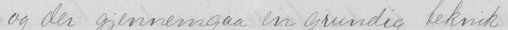og der gjennemgaa en grundig teknik -
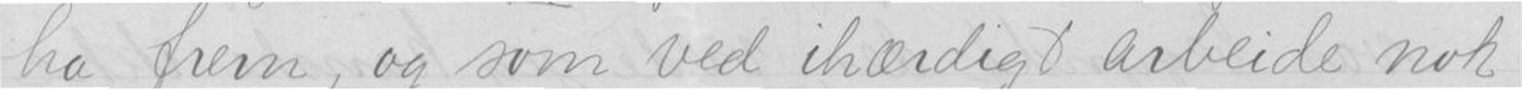ha frem, og som ved ihærdigt arbeide nok
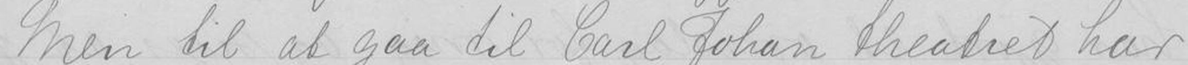Men til at gaa til Carl Johan theatret har
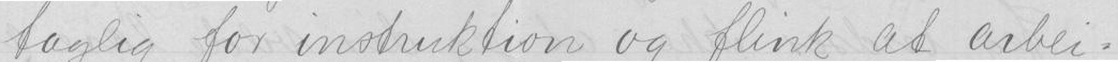taglig for instruktion og flink at arbei-
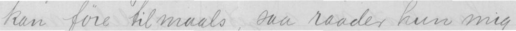kan føre tilmaals, saa raader hun mig
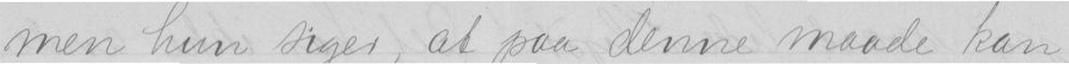men hun siger, at paa denne maade kan
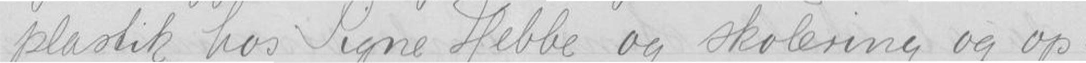plastik hos Signe Hebbe og skolering og op-
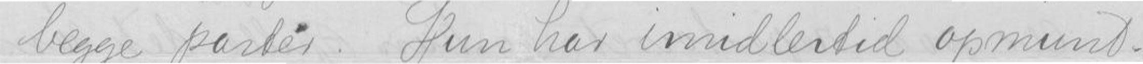begge parter. Hun har imidlertid opmunt-
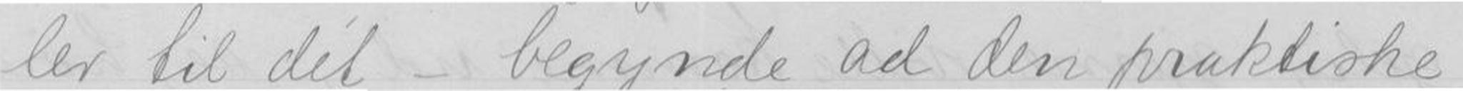ler til det _ begynde ad den praktiske
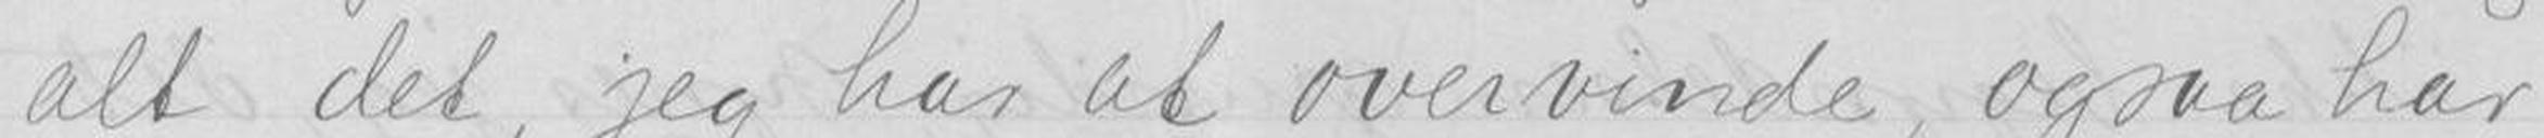alt det, jeg har at overvinde, ogsaa her
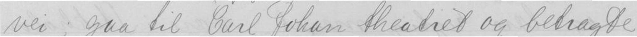vei; gaa til Carl Johan theatret og betragte
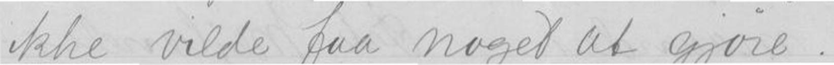ikke vilde faa noget at gjøre.
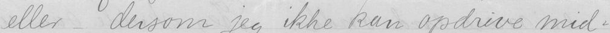eller - dersom jeg ikke kan opdrive mid-
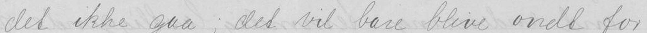det ikke gaa; det vil bare blive ondt for
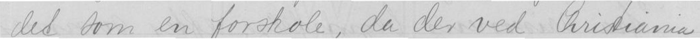det som en forskole, da der ved Christiania
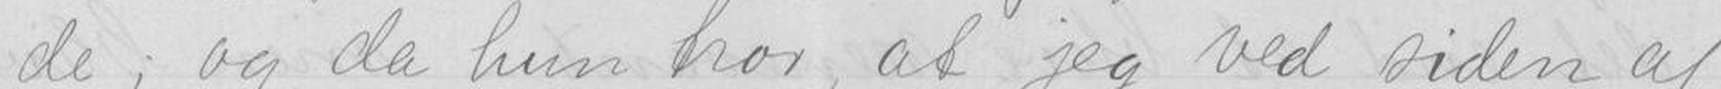de; og da hun tror, at jeg ved siden af
